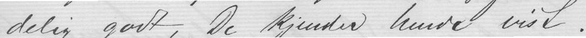delig godt, De kjender hende vist.
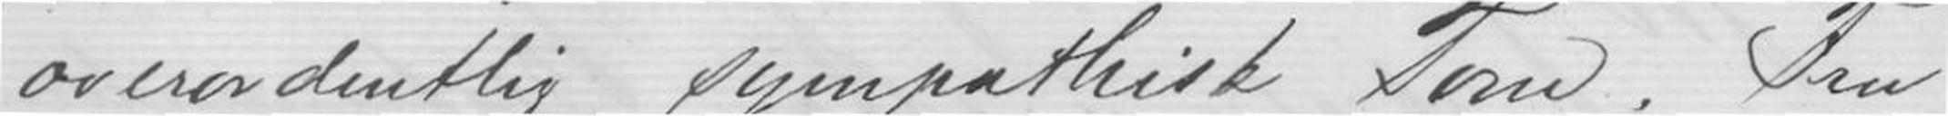overordentlig sympatisk Form. Fru
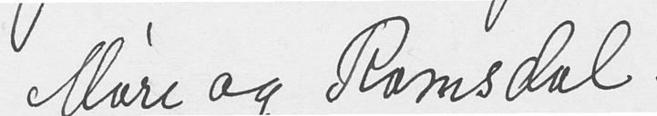Møre og Romsdal
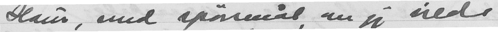Haus, med spørsmål om jeg vilde
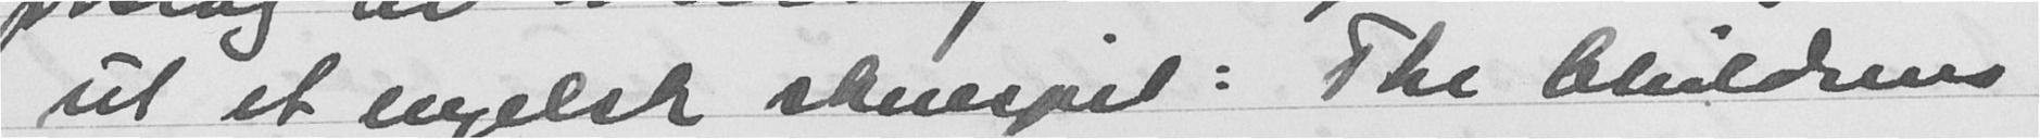ut et engelsk skuespil: The Chuldsens
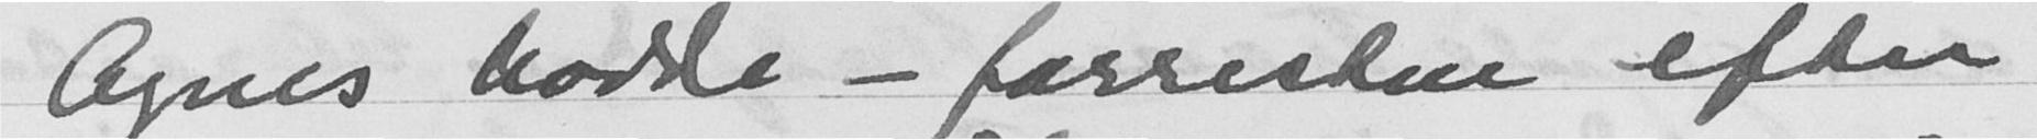Agnes hadde - forresten efter
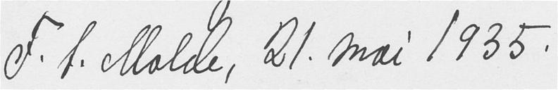P. S. Holse, 21. Mai 1935
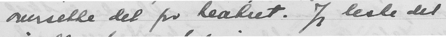oversette det for teatret. Jeg leste det
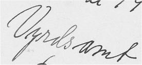Vyrdsamt
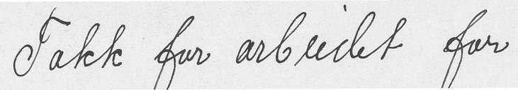Takk fra arbeidet for
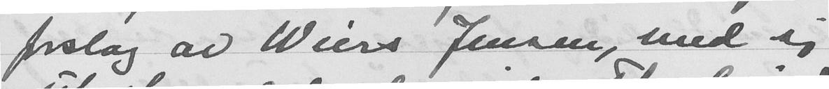forslag av Wiers Jensen, med sig
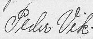Peder Vik
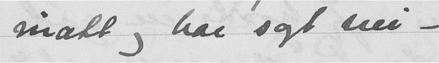inatt og har sagt nei -
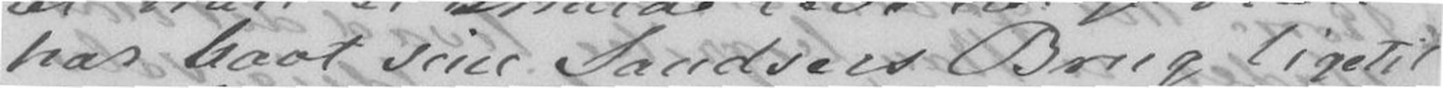har havt sine Sandsers Brug ligetil
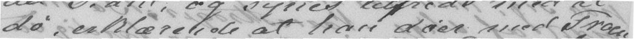dø, erklærende at han døer med Troen
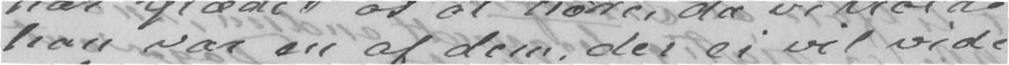han var en af dem, der ei vil vide
